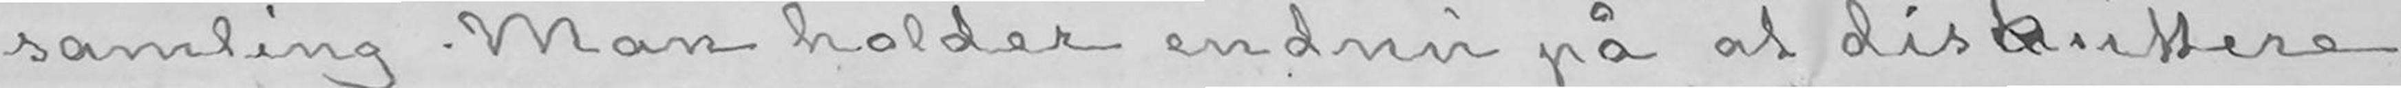samling. Man holder endnu på at diskuttere
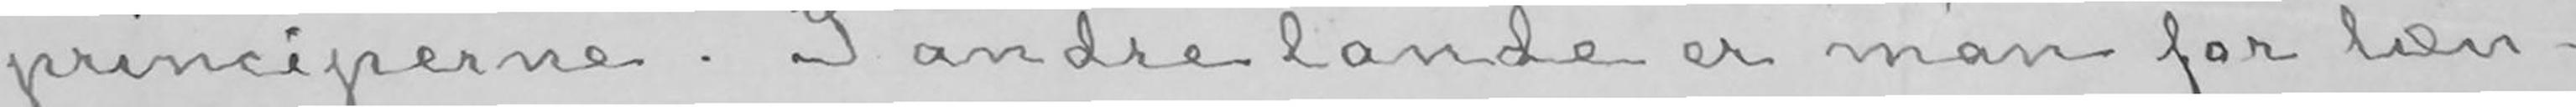principerne. I andre lande er man for læn-
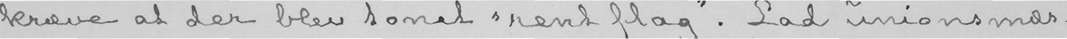kræve at der blev tonet «rent flag». Lad unionsmær-
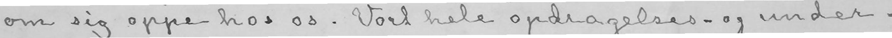om sig oppe hos os. Vort hele opdragelses- og under-
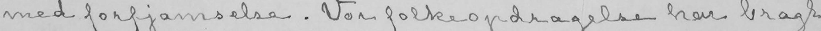med forfjamselse. Vor folkeopdragelse har bragt
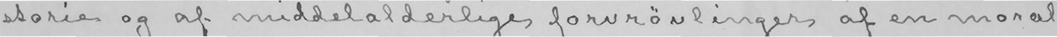storie og af middelalderlige forvrøvlinger af en moral-
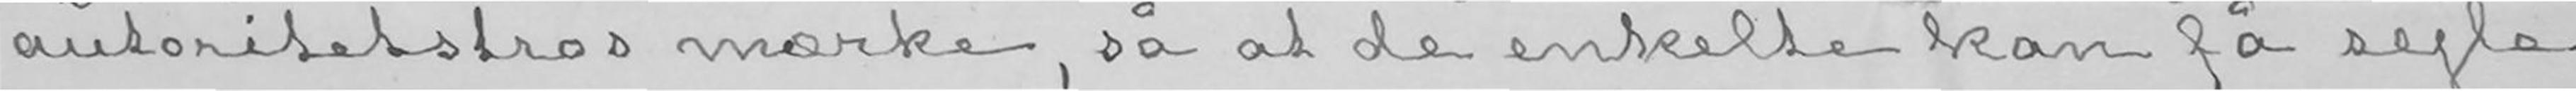autoritetstros mærke, så at de enkelte kan få sejle
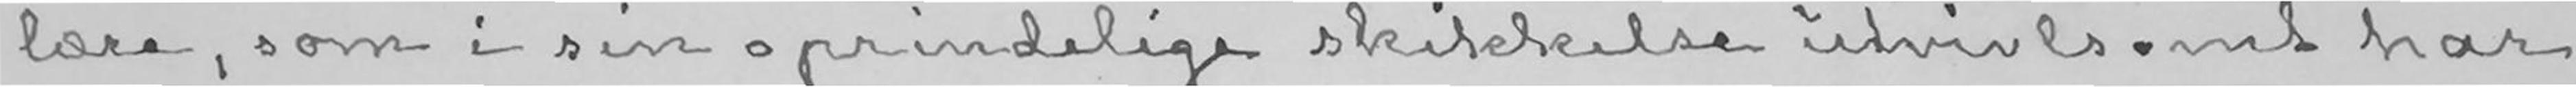lære, som i sin oprindelige skikkelse utvivlsomt har
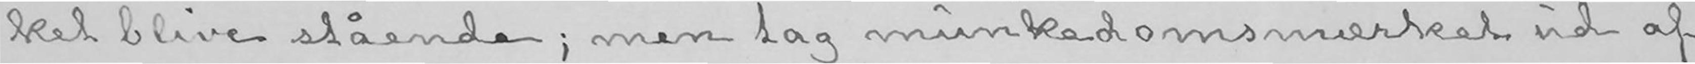ket blive stående; men tag munkedomsmærket ud af
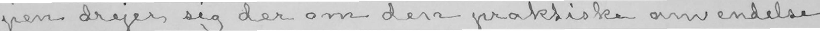pen drejer sig der om den praktiske anvendelse
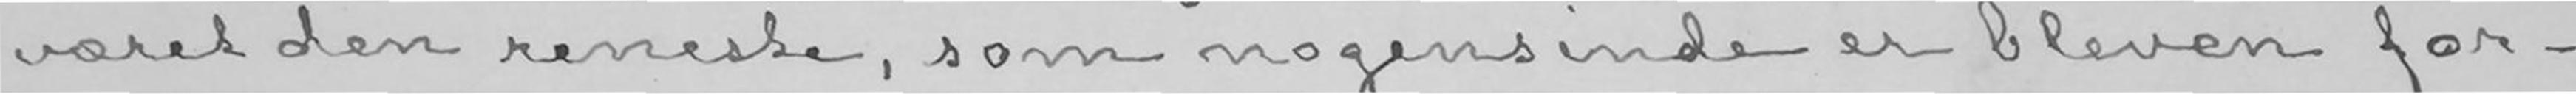været den reneste, som nogensinde er bleven for-
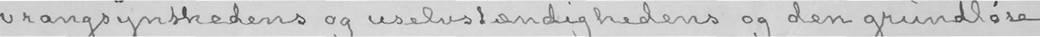vrangsynthedens og uselvstændighedens og den grundløse
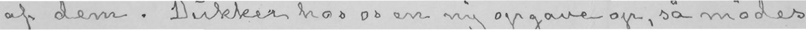af dem. Dukker hos os en ny opgave op, så mødes
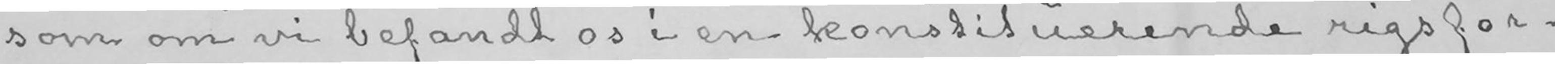som om vi befandt os i en konstituerende rigsfor-
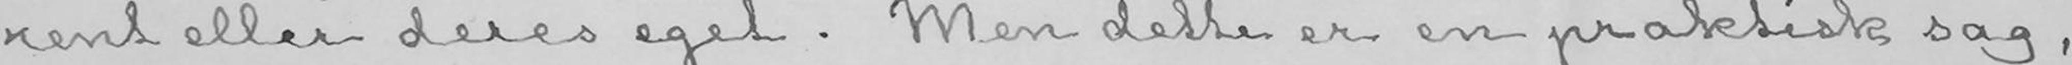rent eller deres eget. Men dette er en praktisk sag,
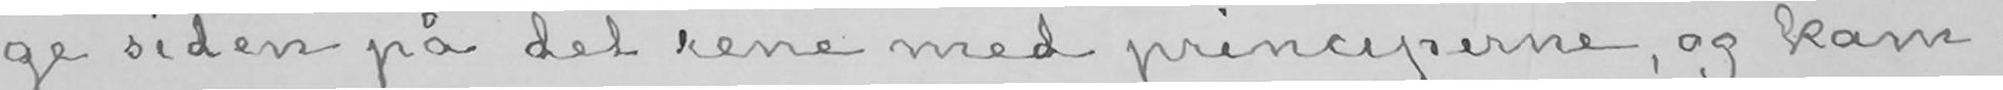ge siden på det rene med principerne, og kam-
